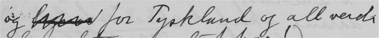og  for Tyskland og all verdi
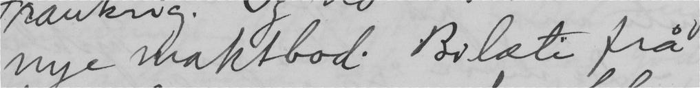nye maktbod. Bilæte frå
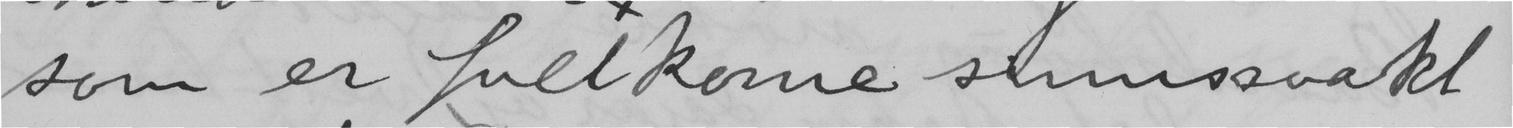som er fullkome sinnssvakt
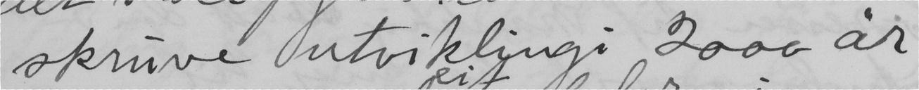skrive utviklingi 2000 år
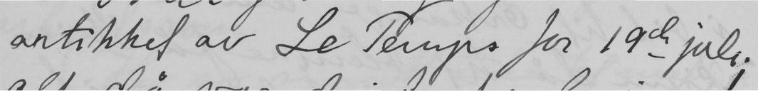artikkel av Le Temps for 19d juli.
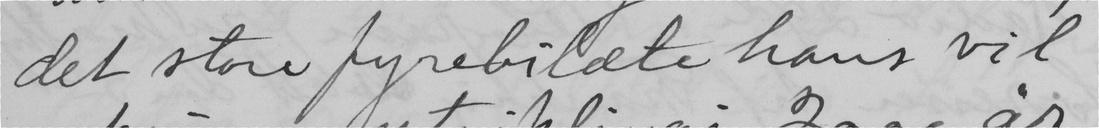det store fyrebilæte hans vil
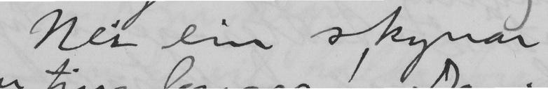Nei ein skynar
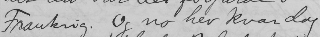Frankrig. Og no hev kvar dag
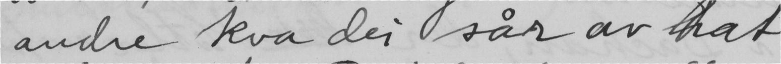andre kva dei sår av hat
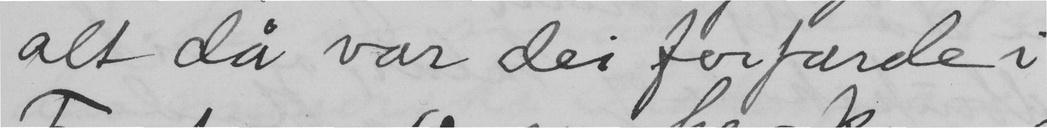alt då var dei forfærde i
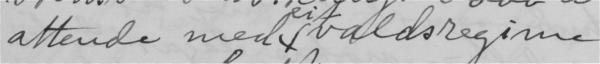attende med eit valdsregime
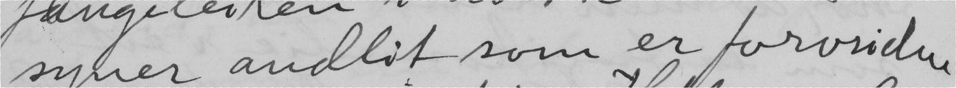syner andlit som er forsvindne
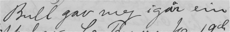Bull gav meg igår ein
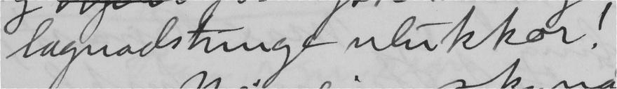lagnadstunge ulukkor!
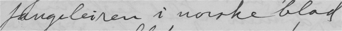fangeleiren i norske blad
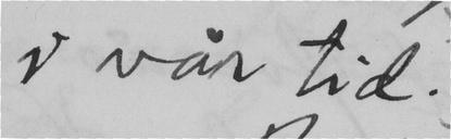i vår tid.
